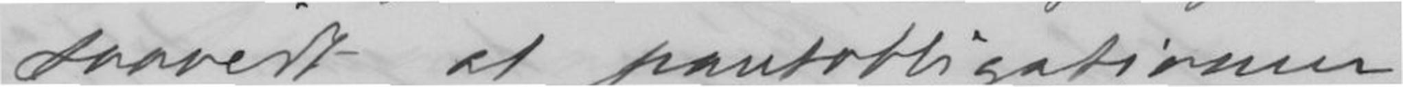saavidt at pantobligationen
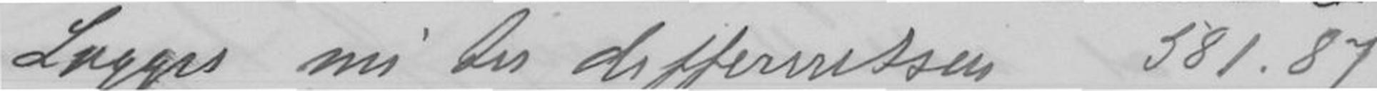Lagges nu til differentsen 581.87
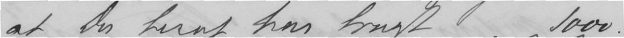at Du heraf har brugt 1000.-
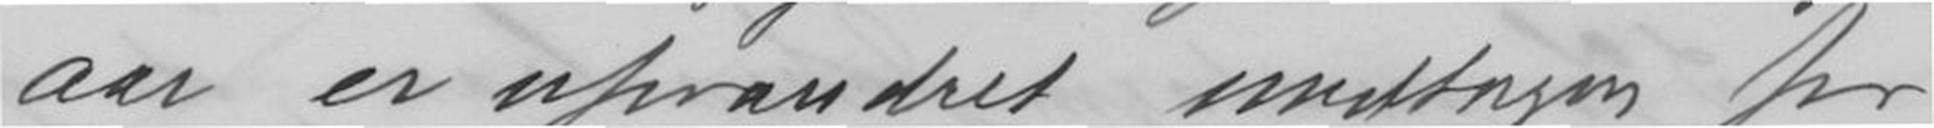aar er uforandret undtagen for
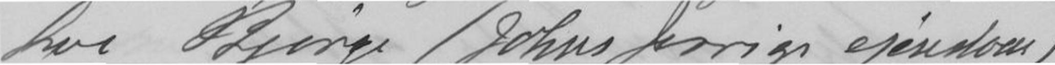hos Bjørge (John forrige ejendom)
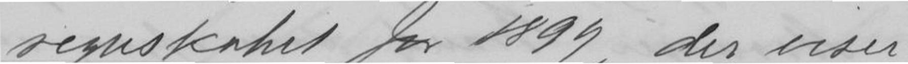regnskabet for 1899, der viser
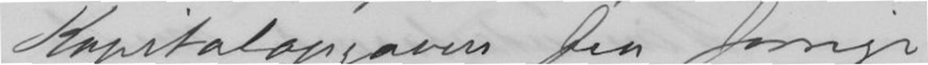Kapitalopgaven for forrige
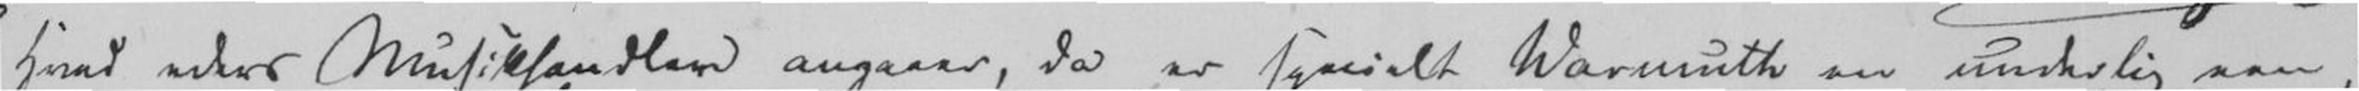Hvad eders Musikhandlen angaar, da er specielt Warmuth en underlig een;
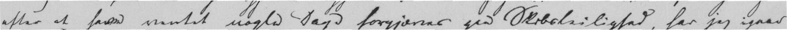efter at have ventet nogle Dage forgjæves pa Skibsleilighed, har jeg igaar
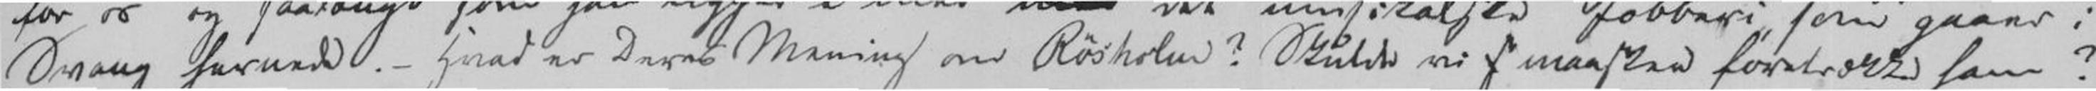Svang hernede. - Hvad er Deres Mening om Røsholm? Skulde vi maaskee foretrække ham?
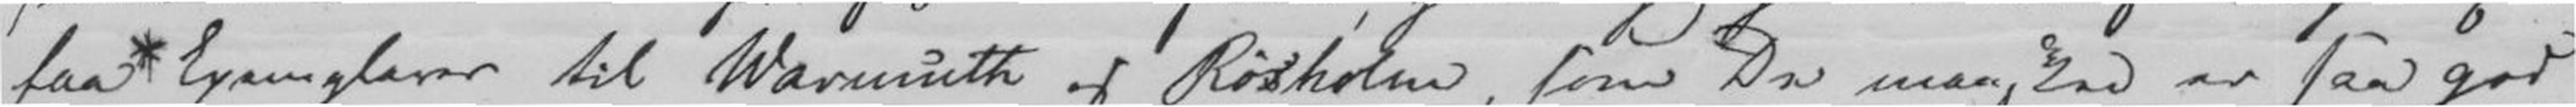faa* Exemplarer til Warmuth og Røsholm, som De maaske er saa God
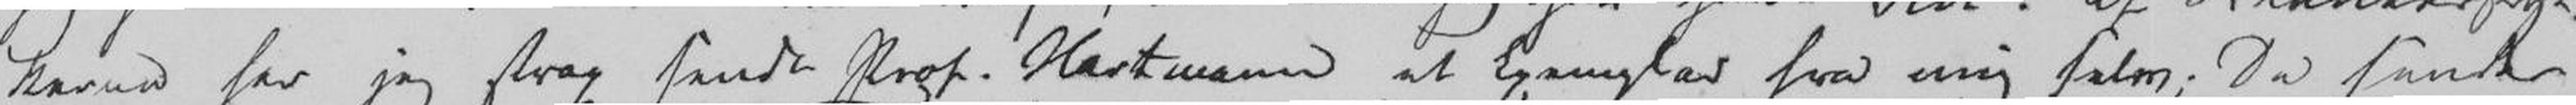kerne har jeg strax sendt Prof. Hartmann et Exemplar fra mig selv; De sender
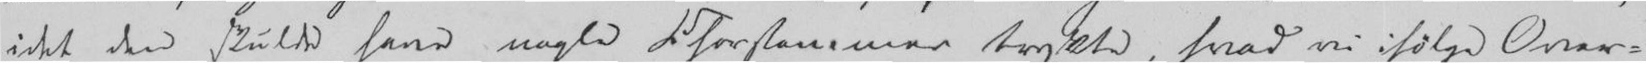idet den skulde have nogle Chorstemmer trygte; hvad vi ifølge Over-
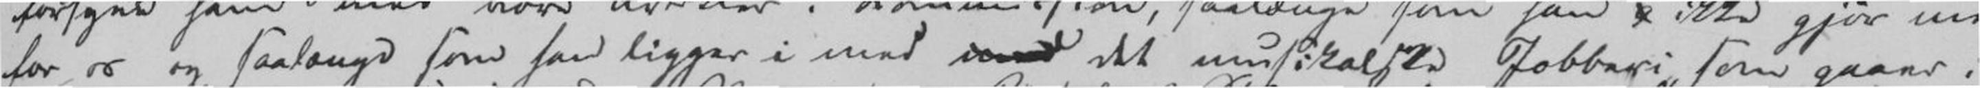for os og saalænge som han ligger i med med det musikalske Jobberi, som gaaer i
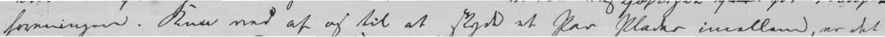foreningen. Kun ved af og til at skyde et Par Plader imellem, er det
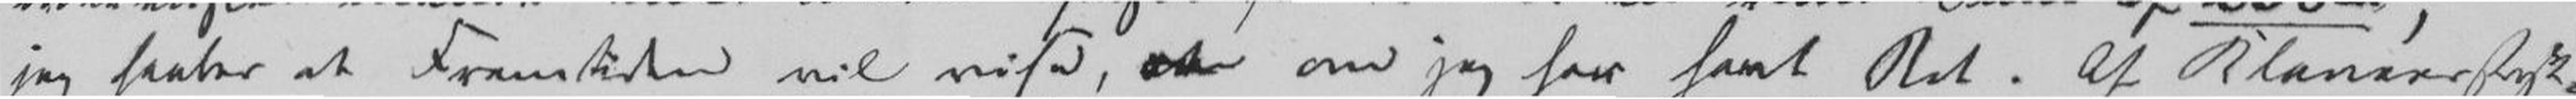jeg haaber at Fremtiden vil vise, den om jeg har havt Ret. Af Klaveerstyk-
